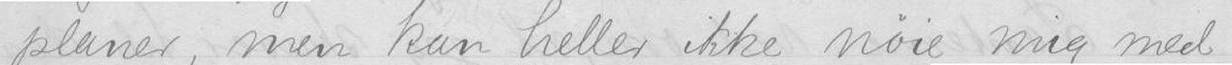planer, men kan heller ikke nøie mig med
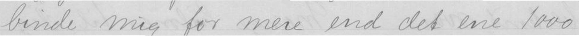binde mig for mere end det ene 1000
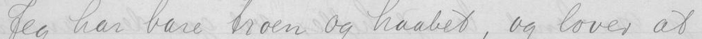Jeg har bare troen og haabet, og lover at
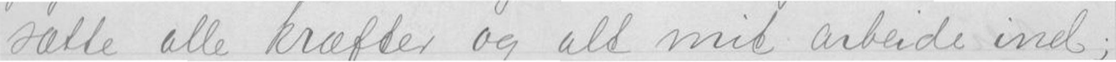sætte alle kræfter og alt mit arbeide ind;
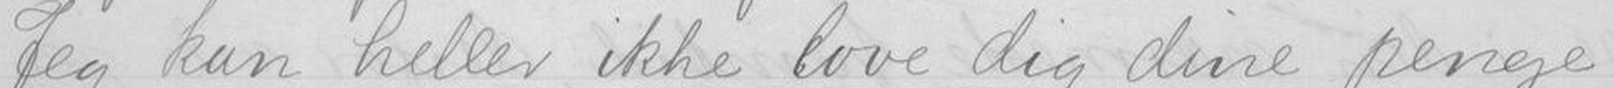Jeg kan heller ikke love dig dine penge
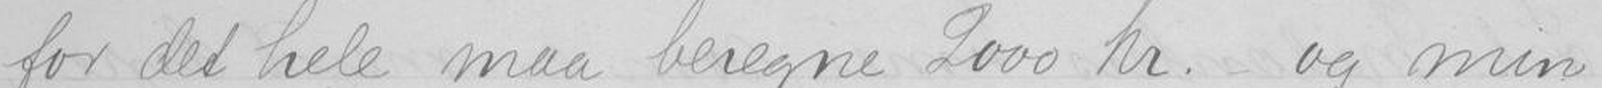for det hele maa beregne 2000 kr. - og min
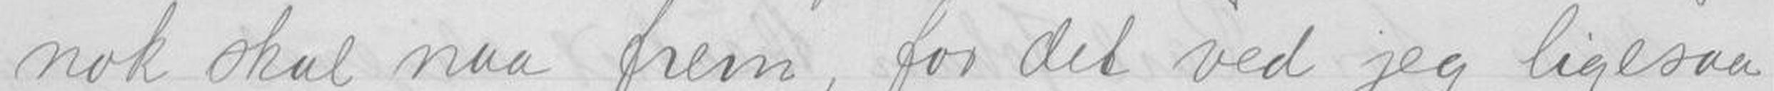nok skal naa frem, for det ved jeg ligesaa
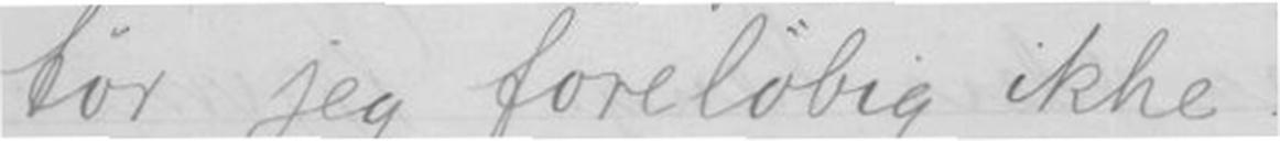tør jeg foreløbig ikke.
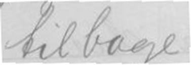tilbage
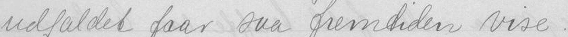udfaldet faar saa fremtiden vise.
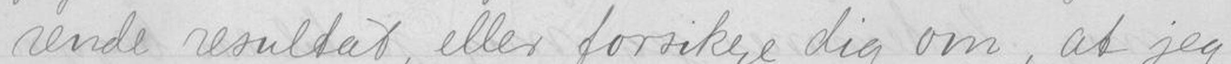rende resultat, eller forsikre dig om, at jeg
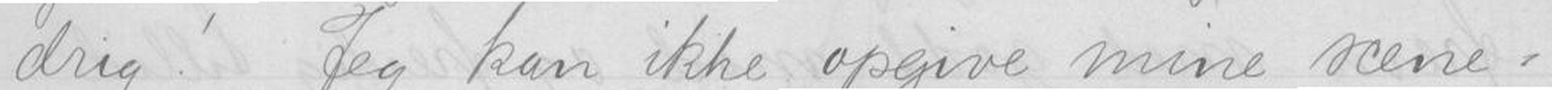drig! Jeg kan ikke opgive mine scene-
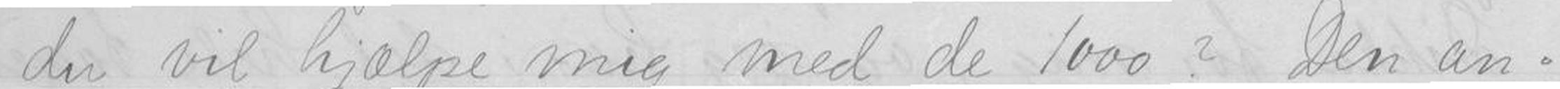du vil hjælpe mig med de 1000? Den an-
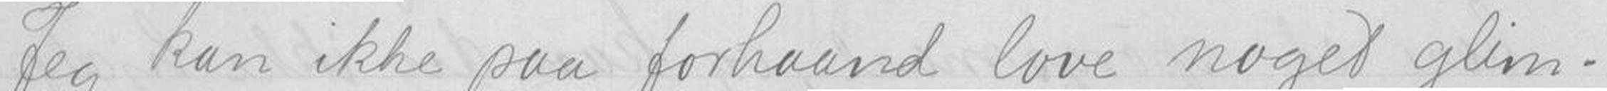Jeg kan ikke paa forhaand love noget glim-
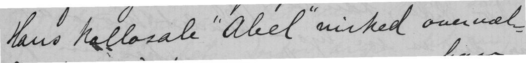Hans Kollosale "Abel" virked overvæl-
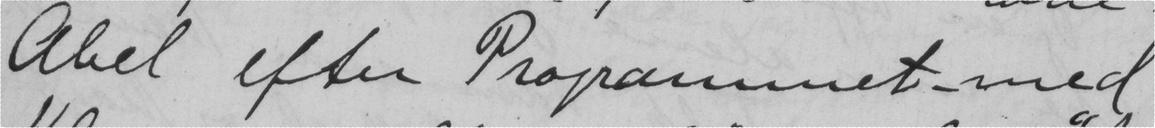Abel efter Programmet - med
